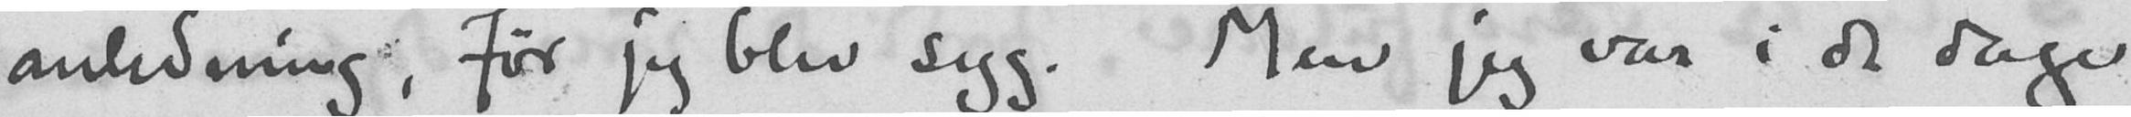anledning, før jeg blev syg. Men jeg var i de dage
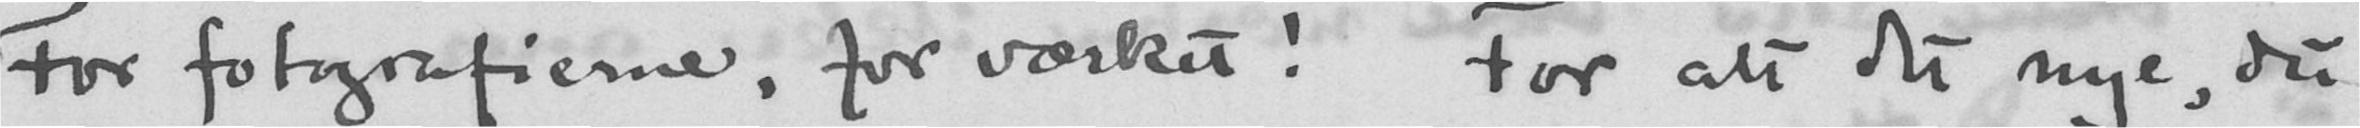For fotografiene, for værket! For alt det nye, du
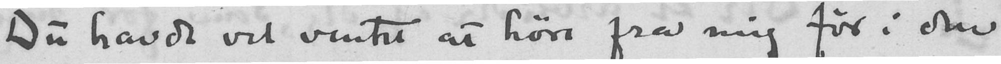Du havde vel ventet at høre fra mig før i den
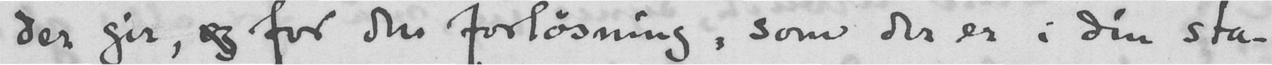der gir, og for den forløsning, som der er i din sta-
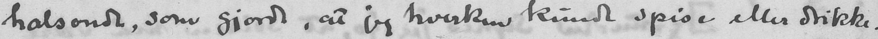halsonde, som gjorde, at jeg hverken kunde spise eller drikke.
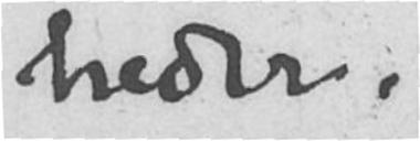heder.
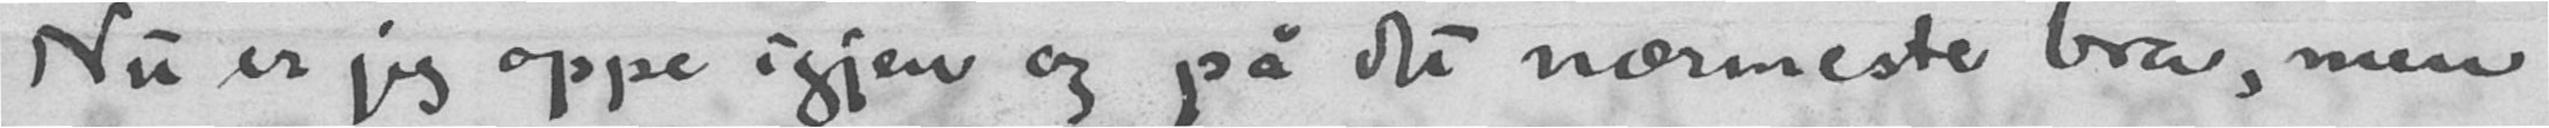Nu er jeg oppe igjen og på det nærmeste bra, men
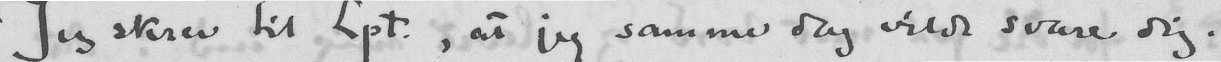Jeg skrev til Lpt., at jeg samme dag vilde svare dig.
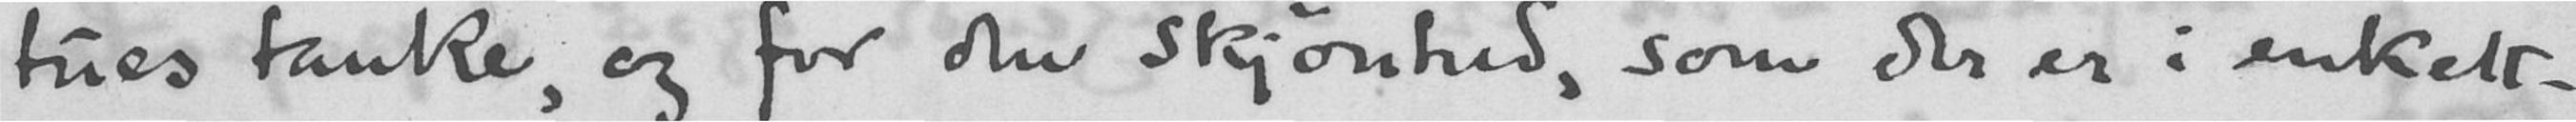tues tanke, og for den skjønhed, som der er i enkelt-
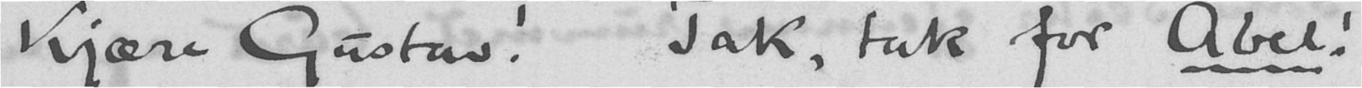Kjære Gustav! Tak, tak for Abel!
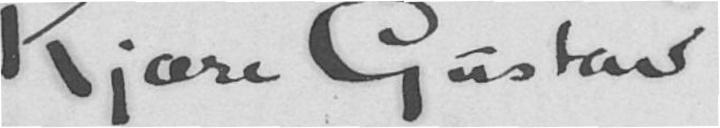Kjære Gustav
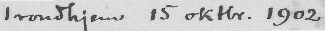Trondhjem 15 oktbr. 1902
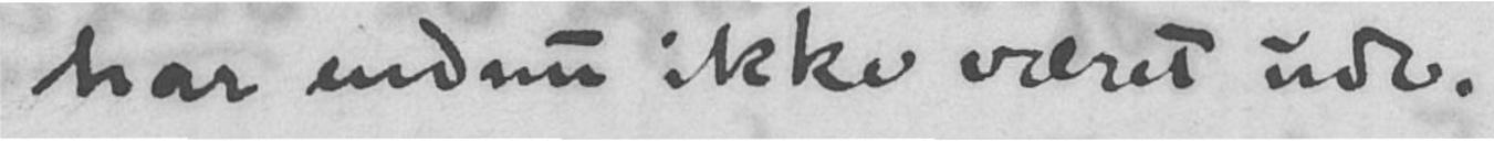har endnu ikke været ude.
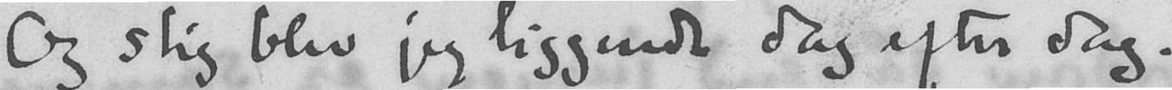Og slig blev jeg liggende dag efter dag.
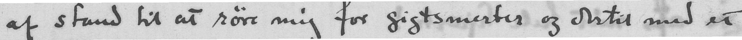af stand til at røre mig for gigtsmerter og dertil med et
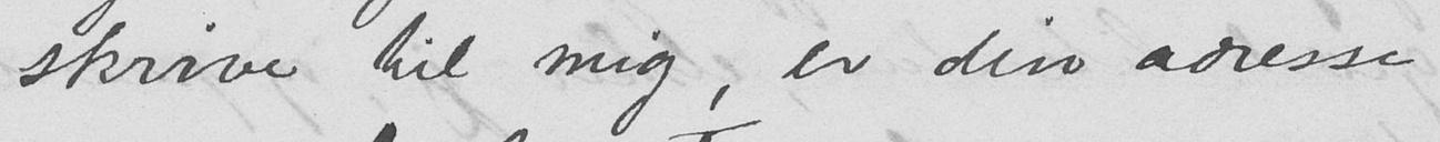skrive til mig, er din adresse
